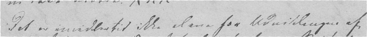Det er imidlertid ikke alene for Udviklingen af
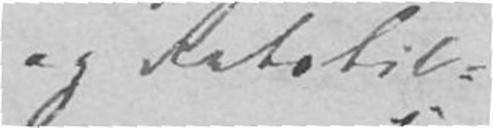og Retstil-
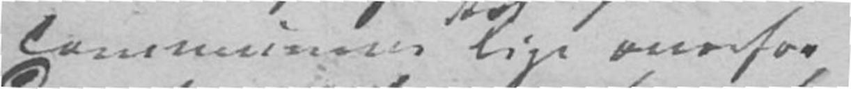Communens lige overfor
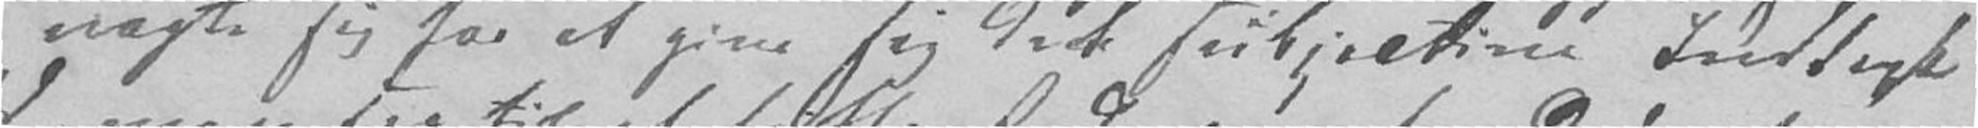vogte sig for at give sig det subjective Indtryk
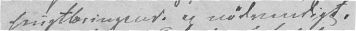frugtbringende og nødvendigt.
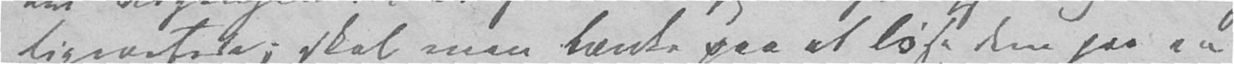ligeartede; skal man tænke paa at løse dem paa en
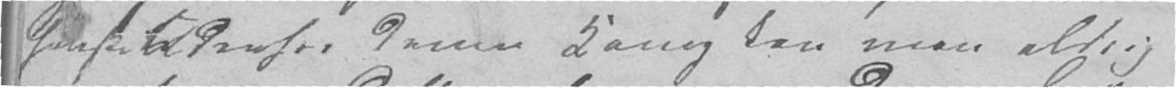Ganske udenfor denne Kamp kan man aldrig
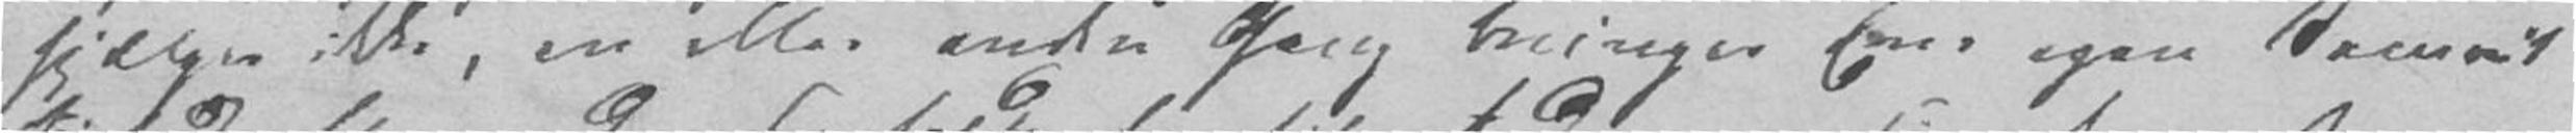hjælper ikke, en eller anden Gang bringer Ens egen Samvit-
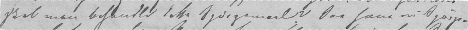skal man behandle dette Spørgsmaal? Saa have vi Spørgs-
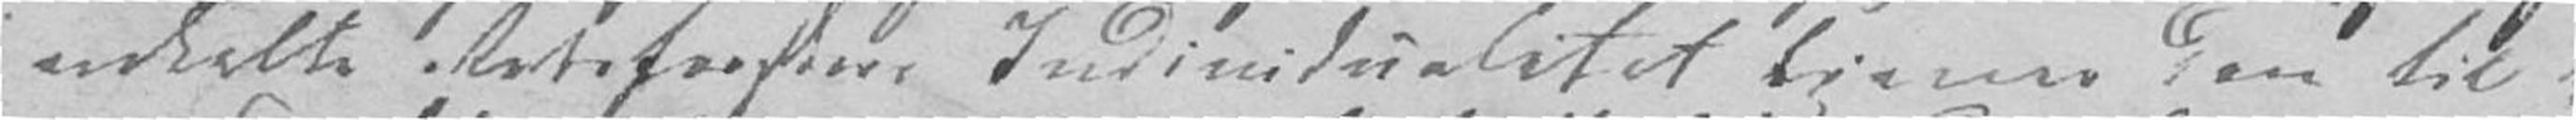enkelte Retsforskers Individualitet tjener den til
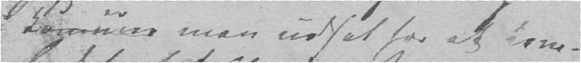man udsat for at kom-
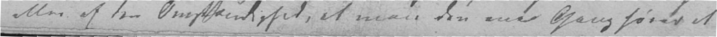eller af den Omstændighed, at man den ene Gang hører et
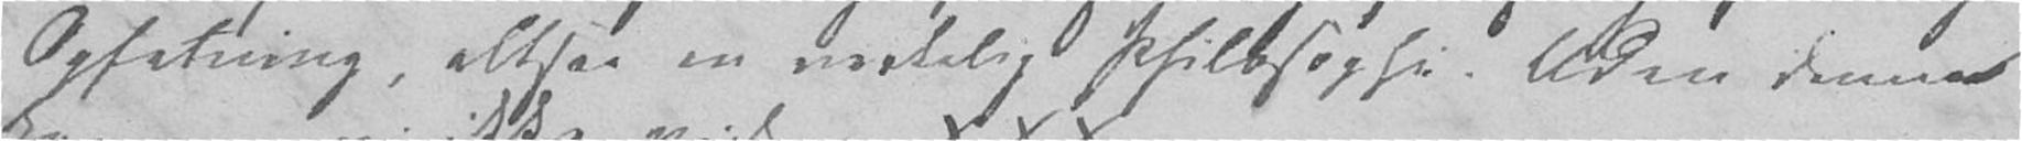Opfatning, altsaa en virkelig Philosophie. Uden denne
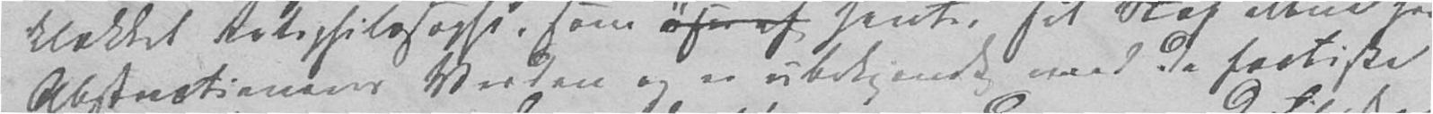Abstractionens Verden og er ubekjendt med de factiske
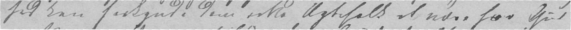hed kan forkynde dem rette Ægtefolk at være for Gud
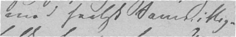med heelst Samvittig-
